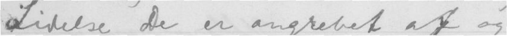Lidelse De er angrebet af og
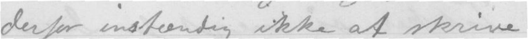derfor instændig ikke at skrive
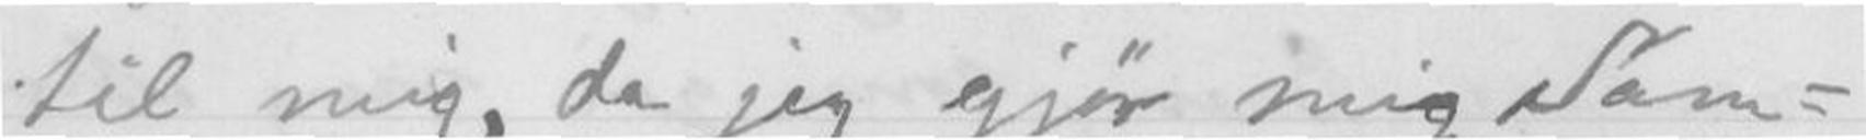til mig, da jeg gjør mig Sam-
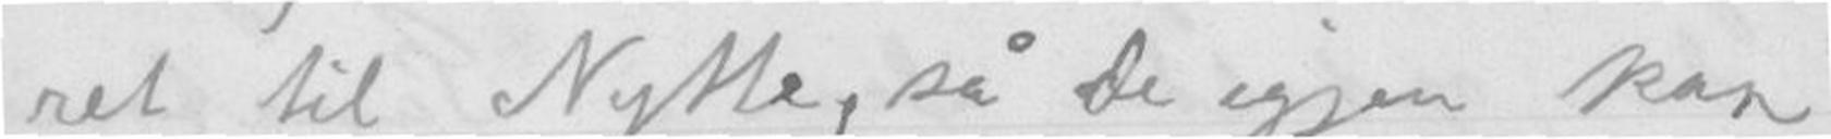ret til Nytte, så De igjen kan
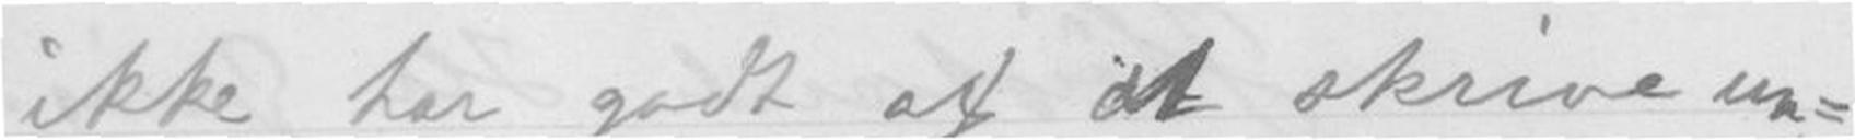ikke har godt af at skrive un-
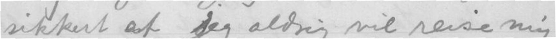sikkert at jeg aldrig vil reise mig
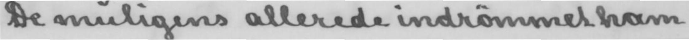De muligens allerede indrømmet ham
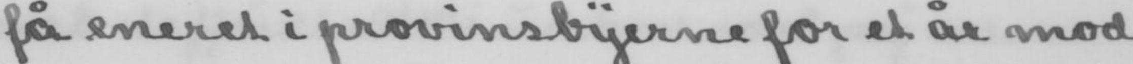få eneret i provinsbyerne for et år mod
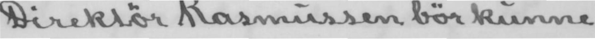Direktør Rasmussen bør kunne
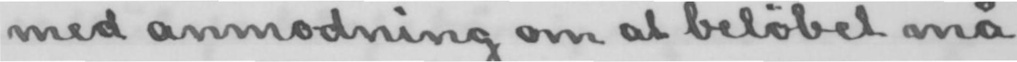med anmodning om at beløbet må
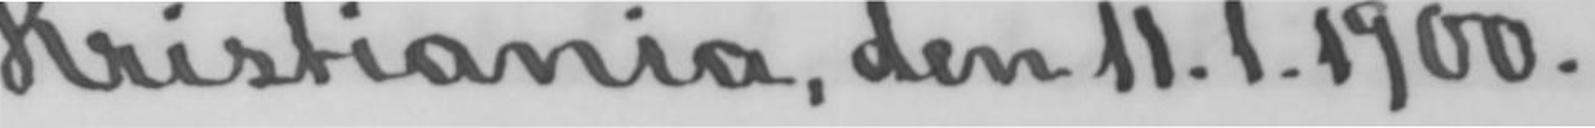Kristiania, den 11.1.1900.
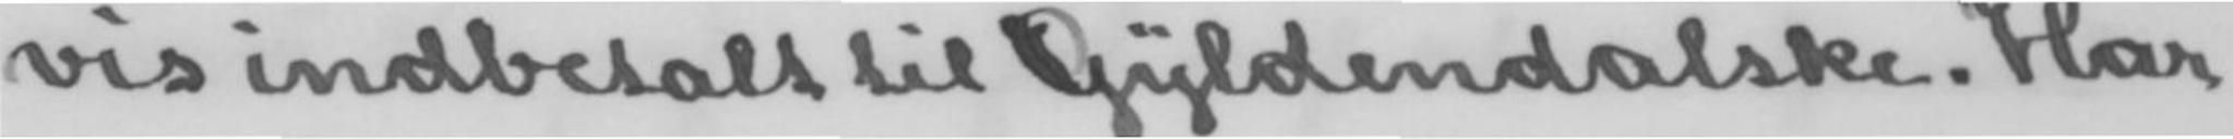vis indbetalt til Gyldendalske. Har
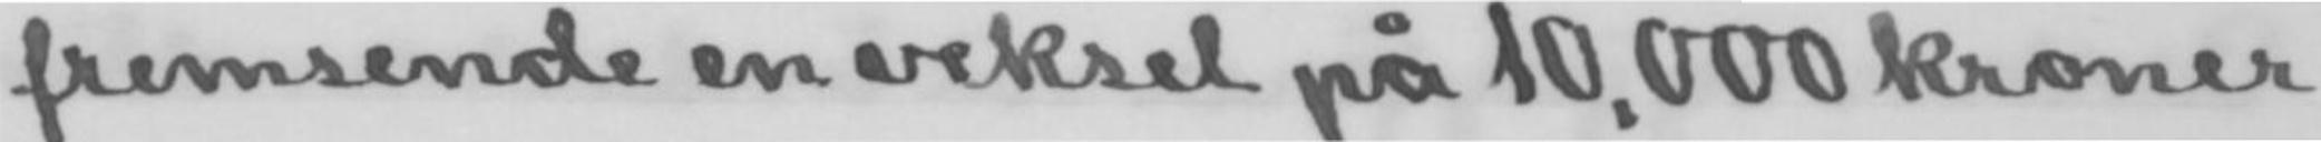fremsende en veksel på 10,000 kroner
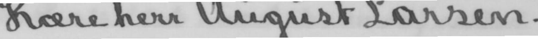Kære herr August Larsen.
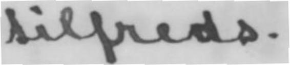tilfreds.
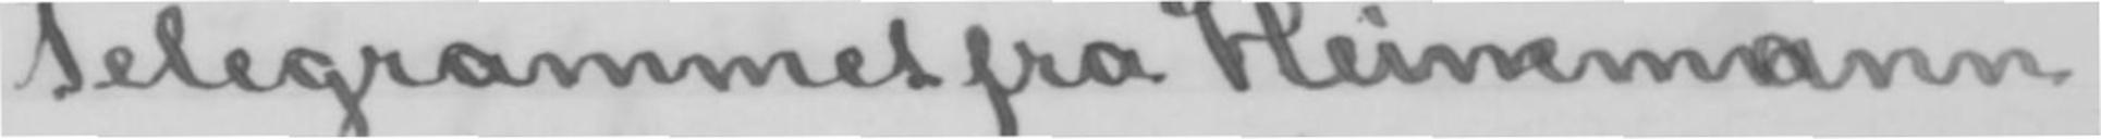Telegrammet fra Heinemnann
